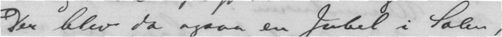Der blev da ogsaa en Jubel i Solen,
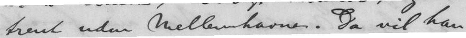trent uden Mellemhavne. Da vil han
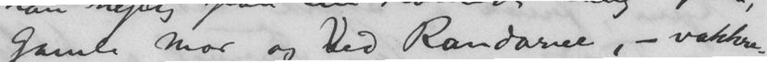Gamle Mor og Ved Rondarne, - vakkre-
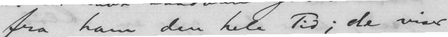fra ham den hele Tid; de viser
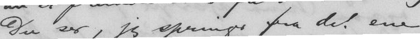- Du ser, jeg springer fra det ene
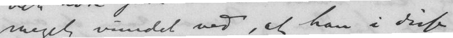meget vundet ved, at han i disse
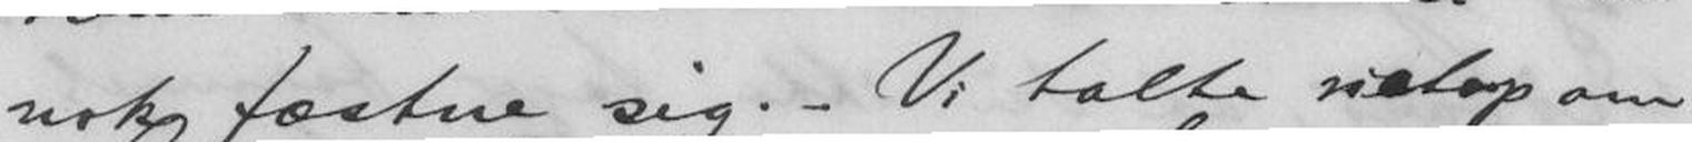nok fæstne sig. - Vi talte netop om
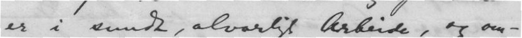er i sundt, alvorligt Arbeide, og om-
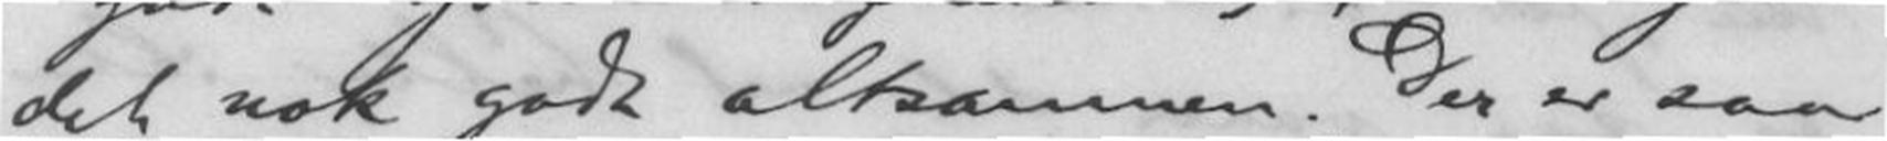det nok godt altsammen. Der er saa
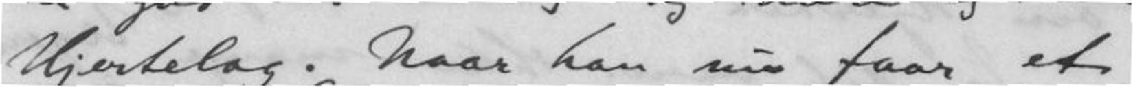Hjertelag. Naar han nu faar et
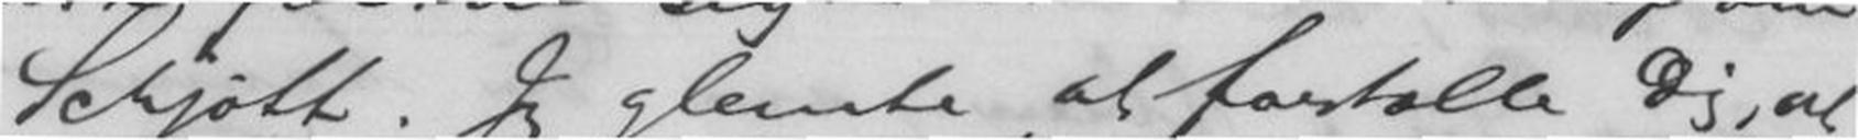Schjøtt. Jeg glemte at fortælle Dig, at
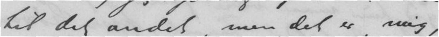til det andet, men det er mig,
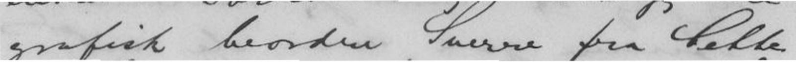grafisk beordre Sverre fra Cette
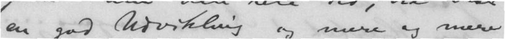en god Udvikling og mere og mere
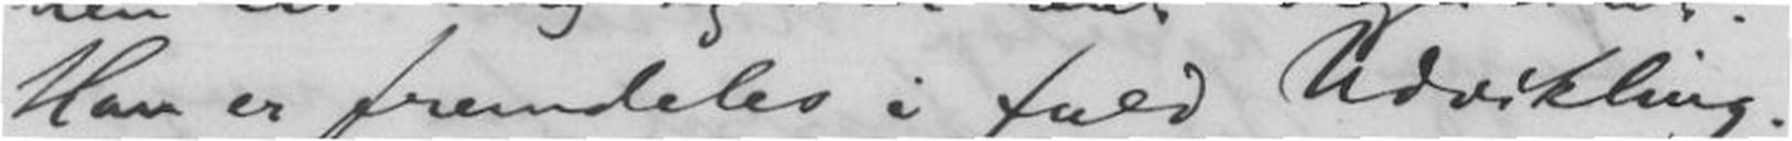Han er fremdeles i fuld Udvikling.
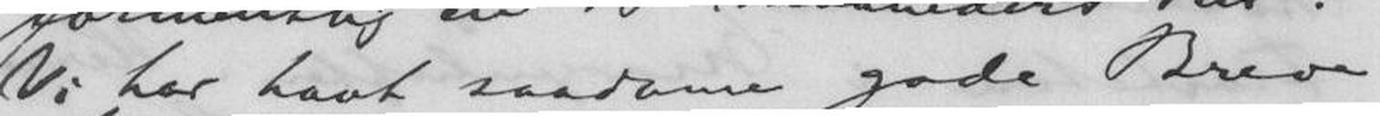Vi har havt saadanne gode Breve
